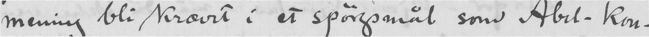mening bli krævet i et spørgsmål som Abel-kon-
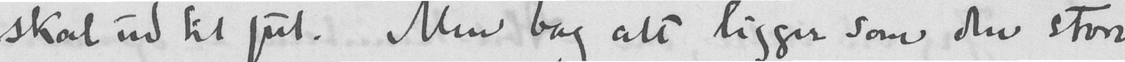skal ud til jul. Men bag alt ligger som den store
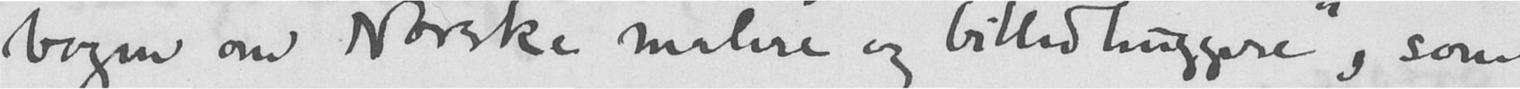bogen om "Norske malere og billedhuggere“, som
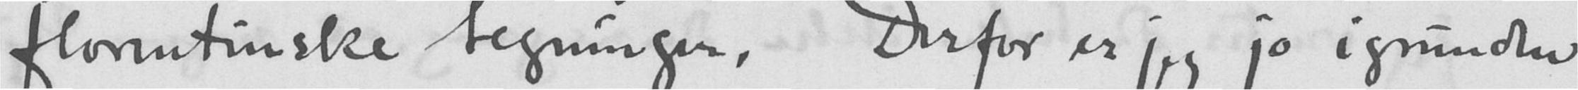florentinske tegninger. Derfor er jeg jo igrunden
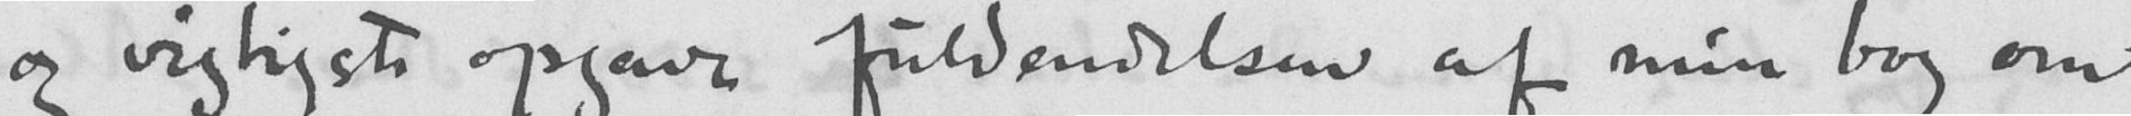og vigtigste opgave fuldendelsen af min bog om
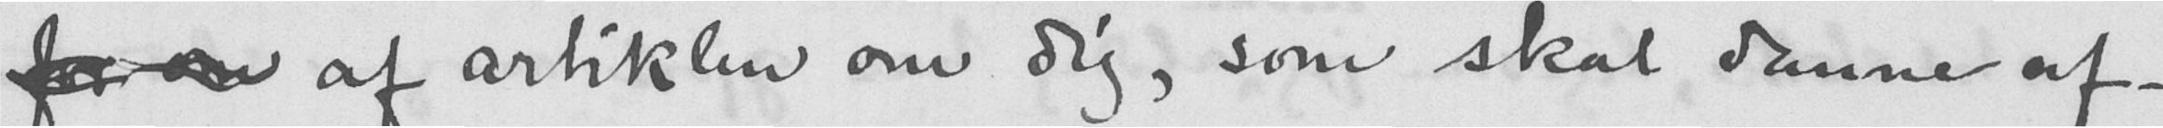for om af artiklen om dig, som skal danne af-
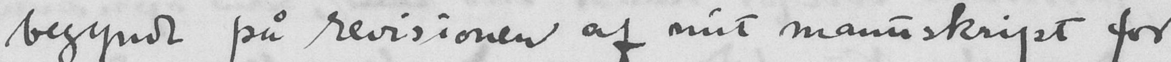begynde på revisionen af mit manuskript for
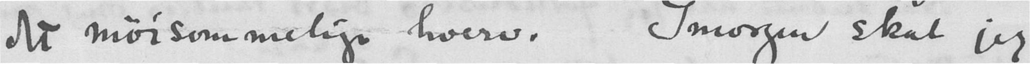det møisommelige hverv. Imorgen skal jeg
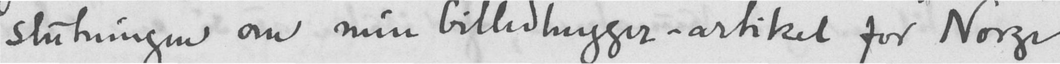slutningen om min billedhugger- artikel for "Norge
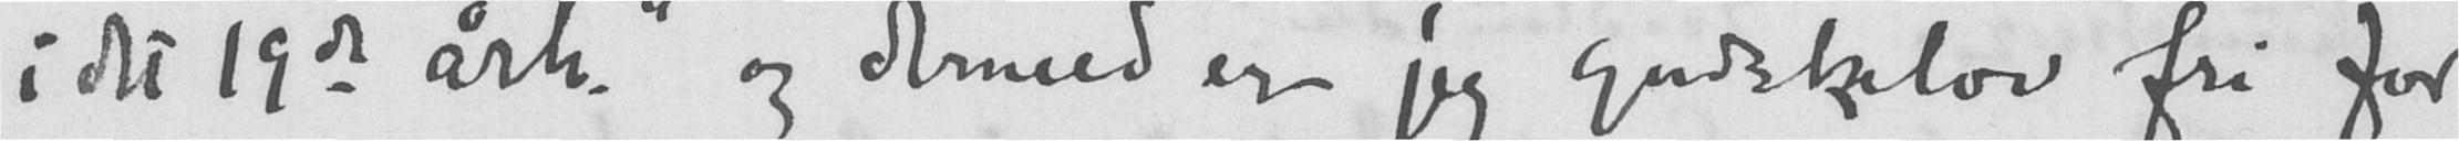i det 19de årh." og dermed er jeg gudskelov fri for
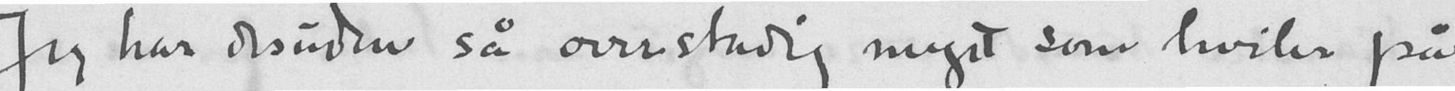Jeg har desuden så overstadig meget som hviler på
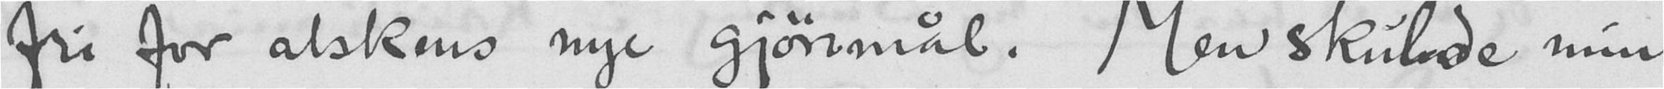fri for alskens nye gjøremål. Men skulde min
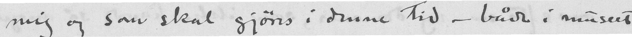mig og som skal gjøres i denne tid - både i museet
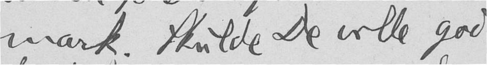mark. Skulde De ville god-
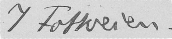7 Fossveien.
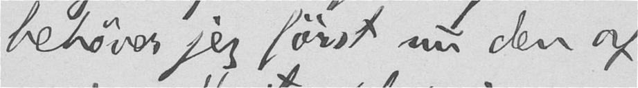behøver jeg først nu den af
